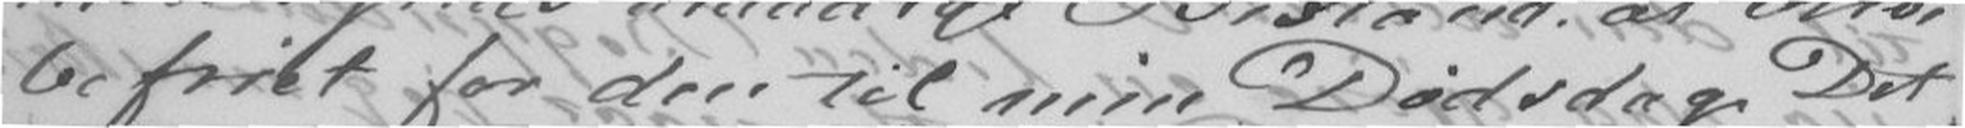befriet for den til min Dødsdag. Det
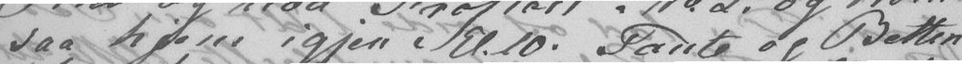saa hjem igjen Kl.10. Tante og Betten
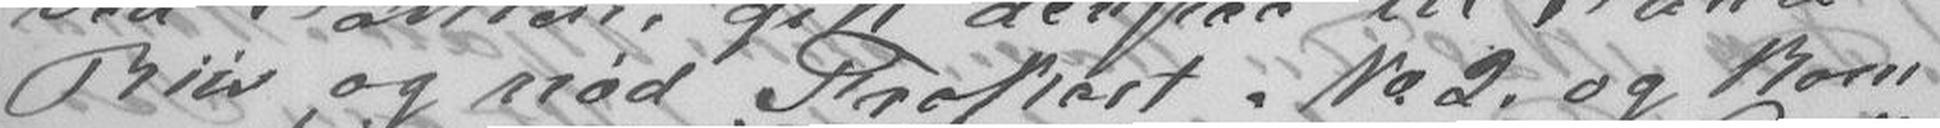Riis og nød Frokost N. 2. og kom
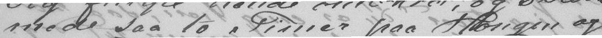mede saa to Timer paa Hougen og
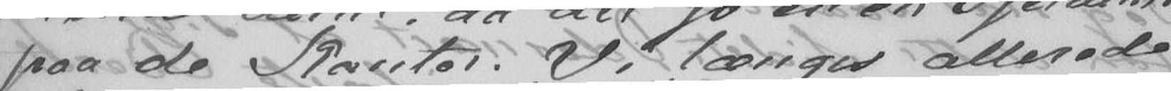paa de Kanter. Vi længes sllerede
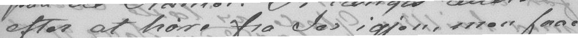efter at høre fra Jer igjen, men faae
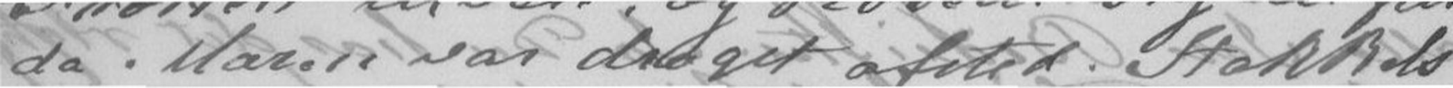da Maren var draget afsted. Stakkels
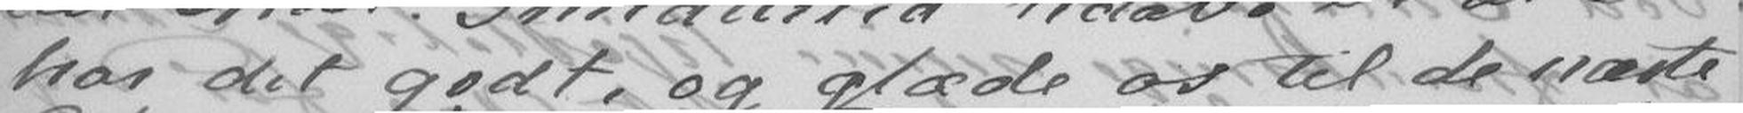har der godt, og glæde os til de næste
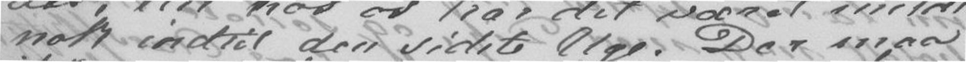nok indtil den sidste Uge. Der maa
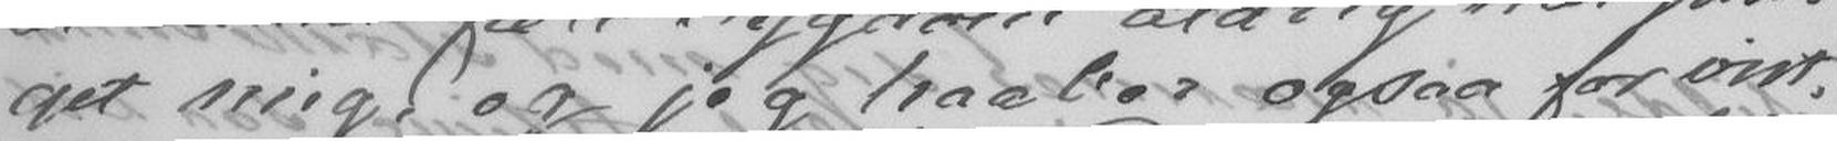get mig, og jeg haaber ogsaa for vist,
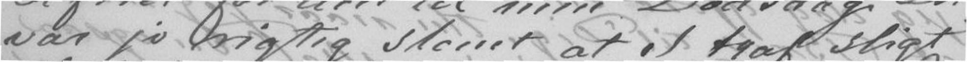var jo rigtig slemt at I traf sligt
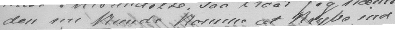den nu kunde komme at krybe ind
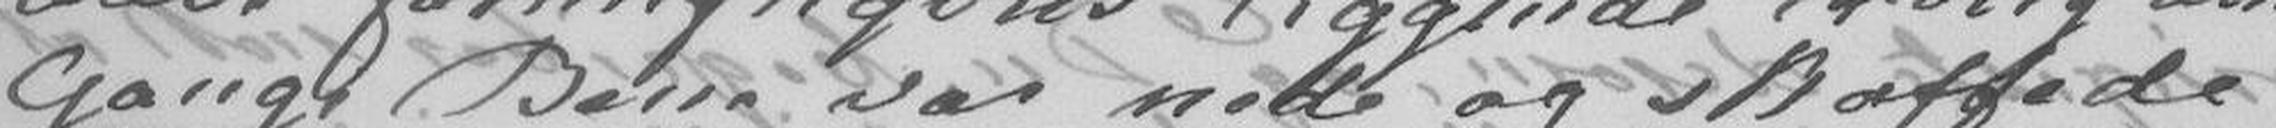Gang, Bene var nede og skaffede
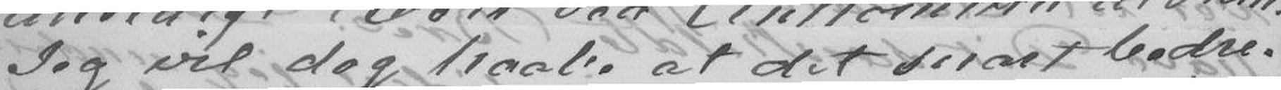Jeg vil dog haabe at det snart bedre-
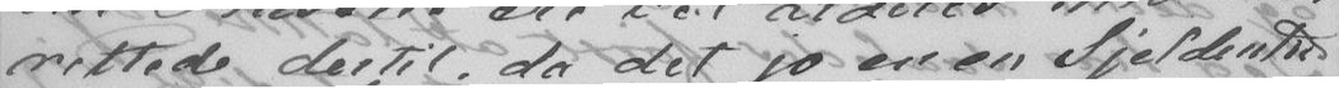rettede dertil, da det jo er en Sjeldenhed
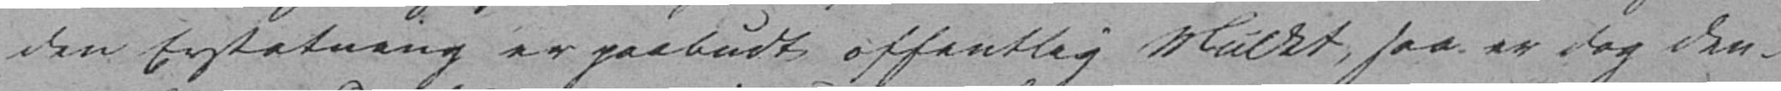den Erstatning er paabudt offentlig Mulkt, saa er dog den-
